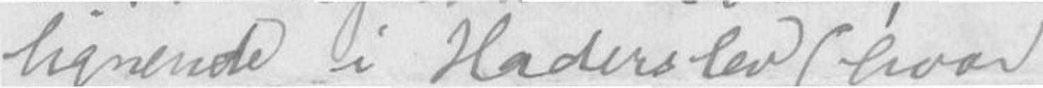lignende i Haderslev?? (hvor
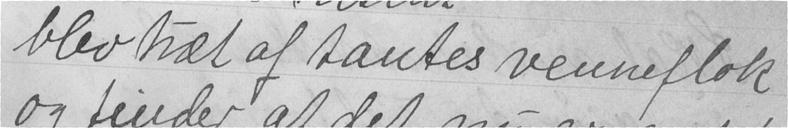blev træt af tantes venneflok
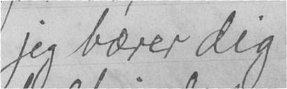jeg bærer dig
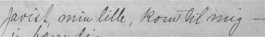Javist, min lille, kom til mig -
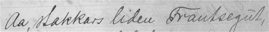Aa, stakkars liden Frantsegut,
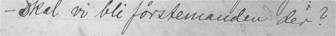- Skal vi bli førstemanden der?
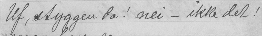Uf, styggen da! nei - ikke det!
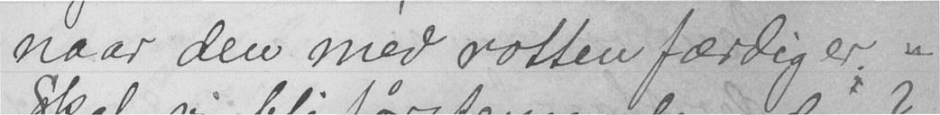naar den med rotten færdig er, -
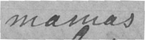mamas
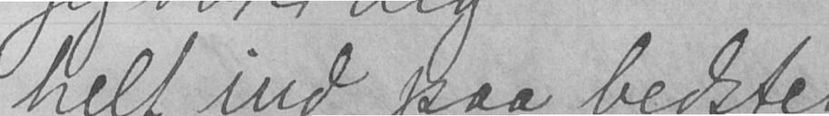helt ind paa bedste
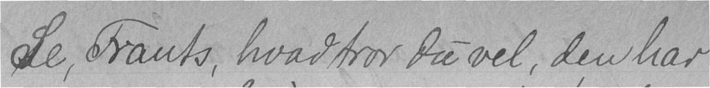Se, Frants, hvad tror du vel, den har
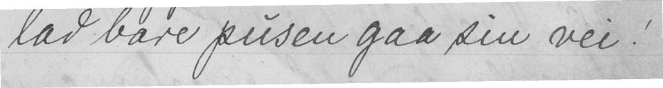lad bare pusen gaa sin vei!
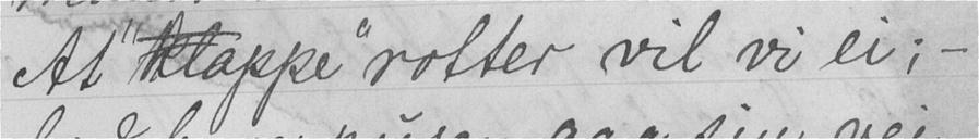At "klappe" rotter vil vi ei; -
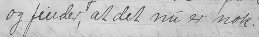og finder, at det nu er nok!
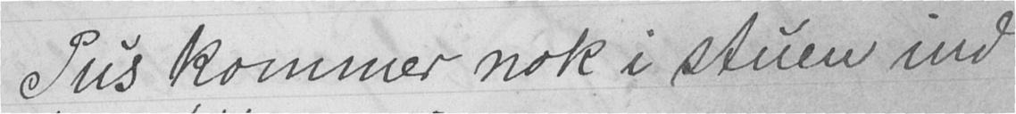Pus kommer nok i stuen ind
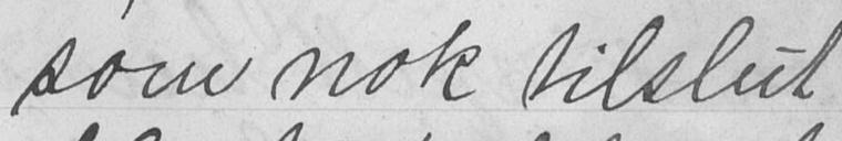som nok tilslut
